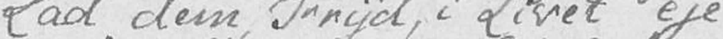Lad dem Fryd i Livet eje
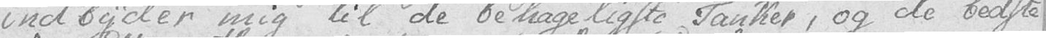indbyder mig til de behageligste Tanker, og de bedste
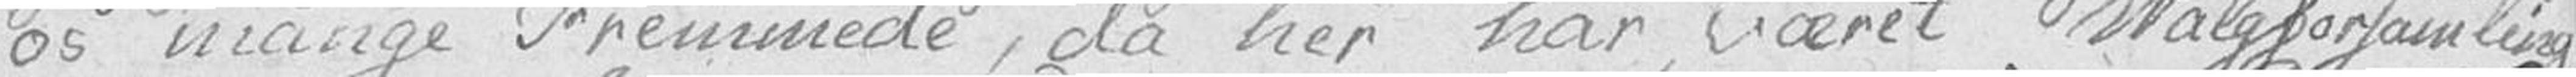os mange Fremmede, da her har været Valgforsamling,
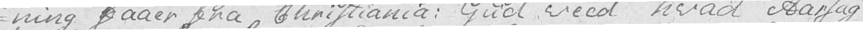-ning faaer fra Christiania: Gud veed hvad Aarsag
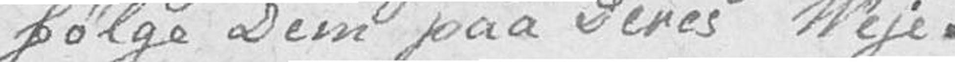følge Dem paa Deres Veje.
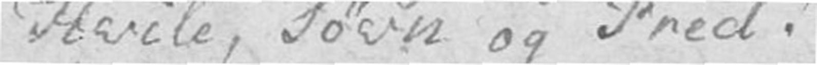Hvile, Søvn og Fred!
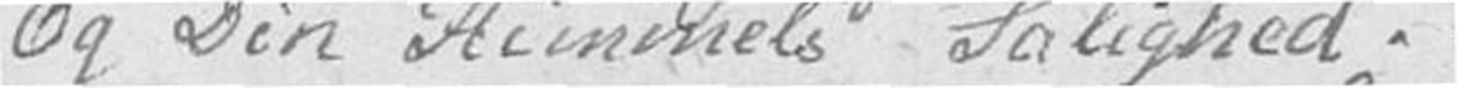Og Din Himmels Salighed. –
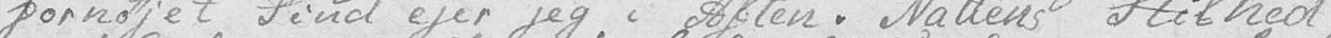fornøjet Sind ejer jeg i Aften. Nattens Stilhed
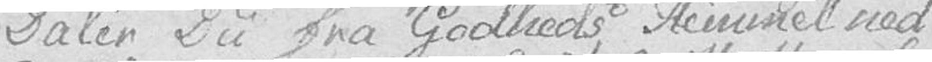Daler Du fra Godheds Himmel ned
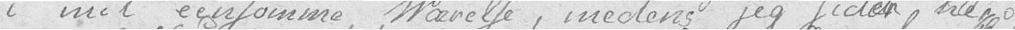i mit eensomme Værelse, medens jeg sider her
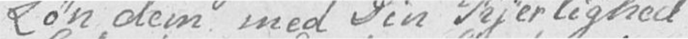Løn dem med Din Kjerlighed
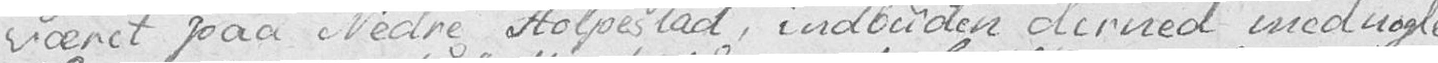været paa Nedre Stolpestad, indbuden derned med nogle
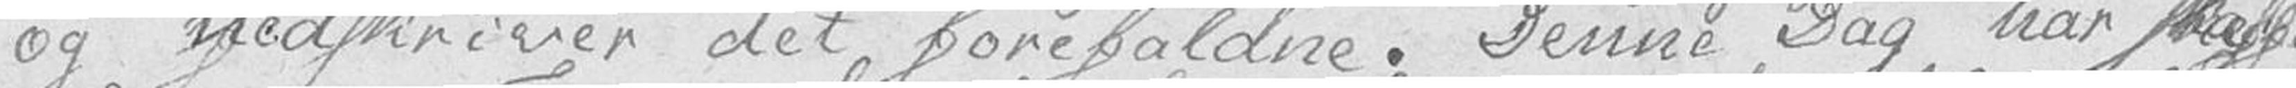og nedskriver det forefaldne. Denne Dag har skænket
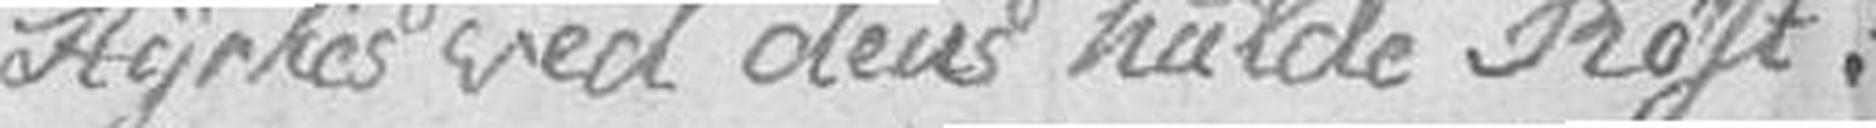Styrkes ved dens hulde Røst!
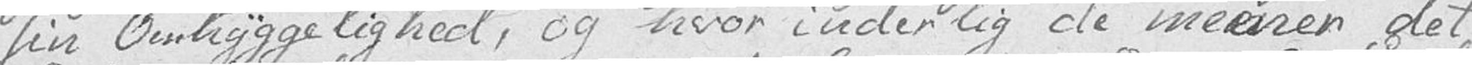sin Omhyggelighed, og hvor inderlig de meener det
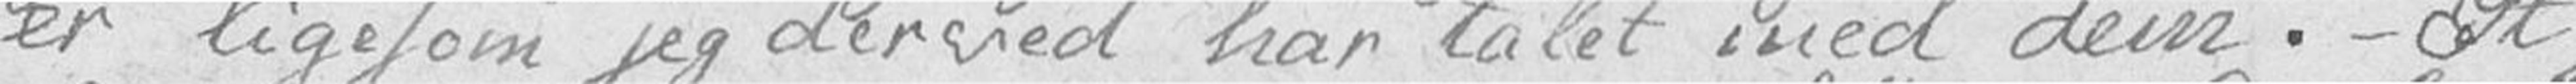er ligesom jeg derved har talet med dem. – Et
